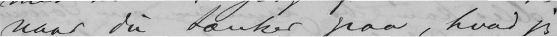naar Du tænker paa, hva jeg
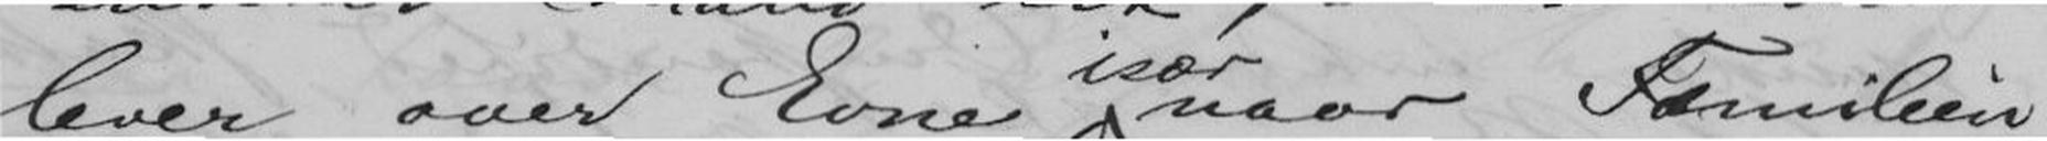lever over Evne naar Familien
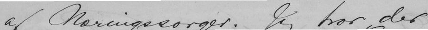af Næringssorger. Jeg tror, der
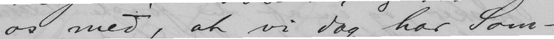os med, at vi dog har Som-
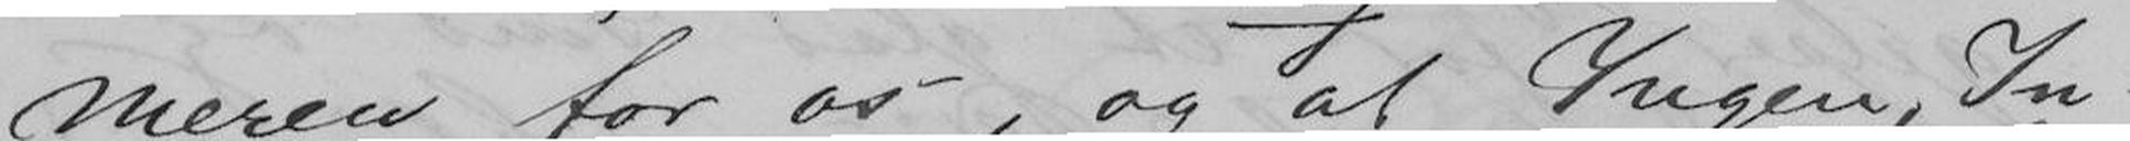meren for os, og at Ingen, In-
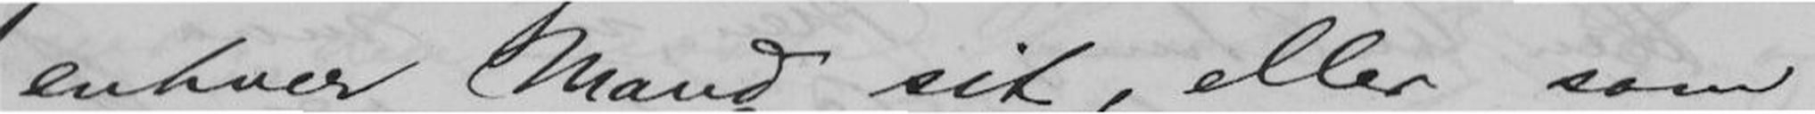enhver Mand sit, eller som
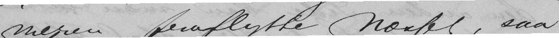meren fraflytte Næsset, saa
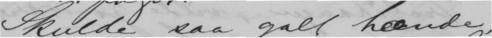Skulde saa galt hænde,
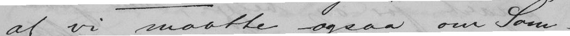at vi maatte ogsaa om Som-
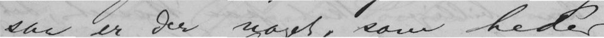saa er der noget, som heder
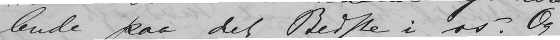lende paa det Bedste i os. Og
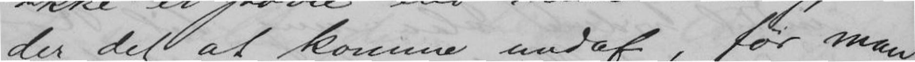der det at komme undaf, før man
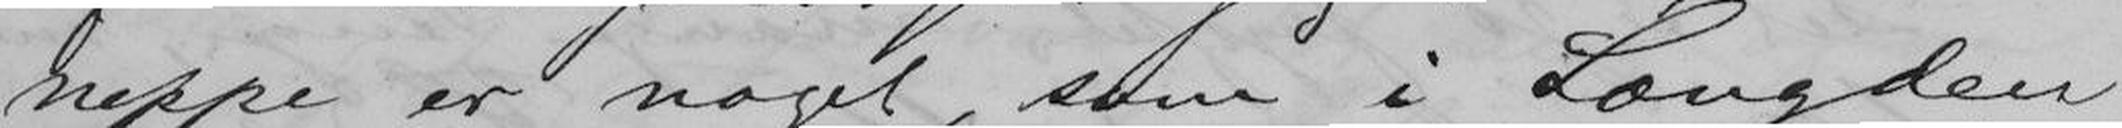neppe er noget, som i Længden
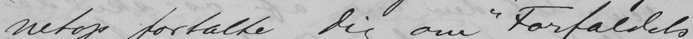netop fortalte Dig om "Forfaldets
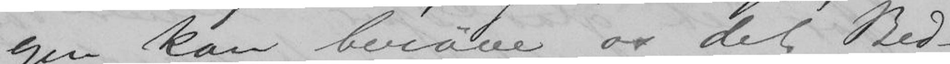gen kan berøve os det Bed-
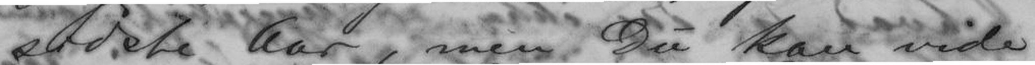sidste Aar, men Du kan vide
